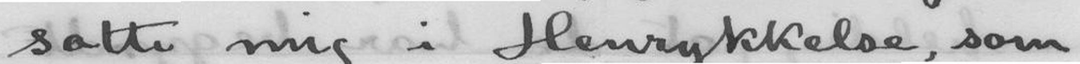satte mig i Henrykkelse, som
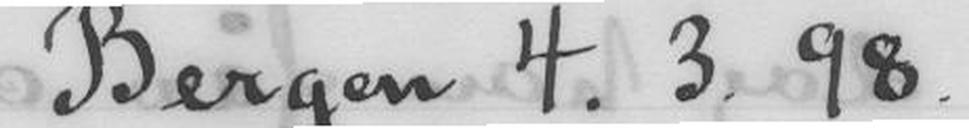Bergen 4.3.98.
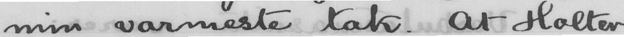min varmeste tak. At Holter
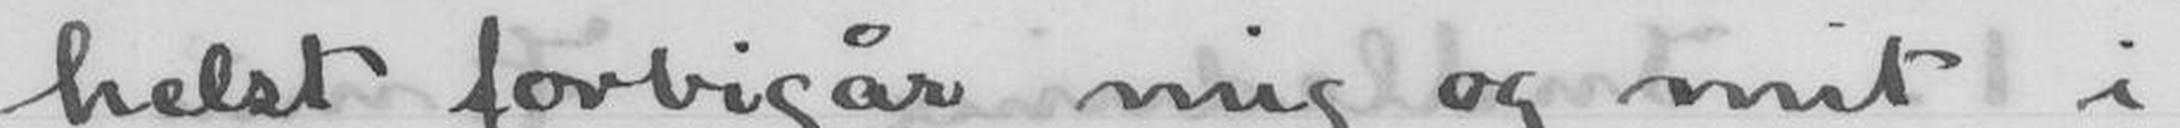helst forbigår mig og mit i
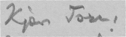Kjære Tore,
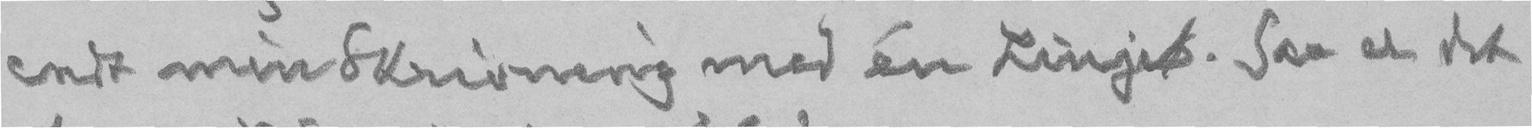endt min Skrivning med en Linjes. Saa er det
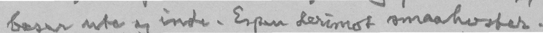baser ute og inde. Espen derimot smaahoster.
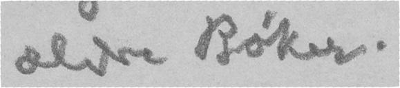ældre Bøker.
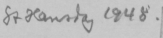St Hansdag 1948.
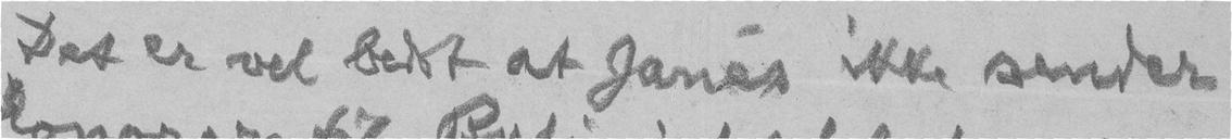Det er vel bedst at Janés ikke sender
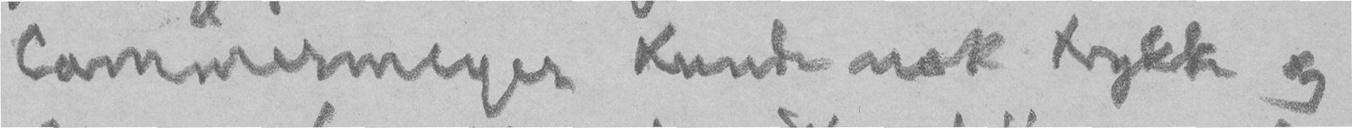Cammermeyer kunde nok trykke og
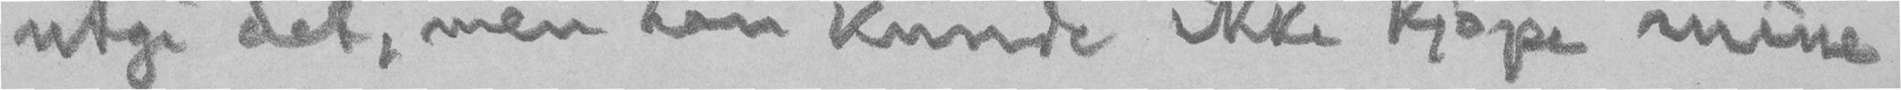utgi det, men han kunde ikke kjøpe mine
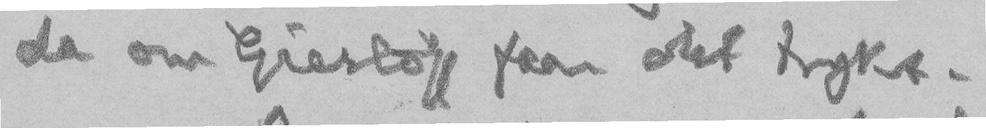da om Gierløff faar det trykt.
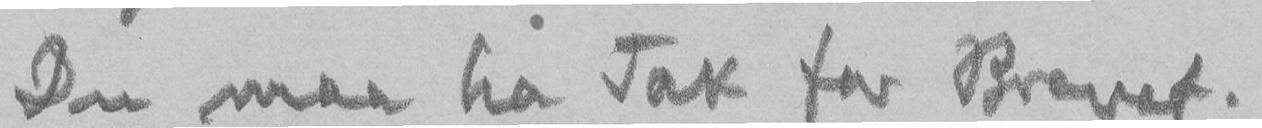Du maa ha Tak for Brevet.
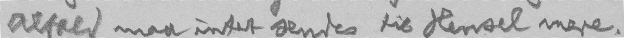alfald maa intet sendes til Hensel mere.
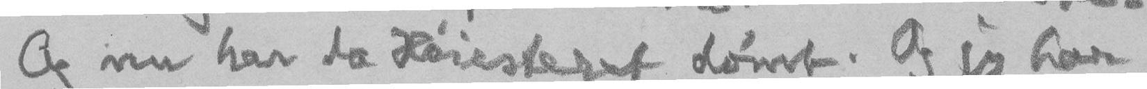Og nu har da Høiesteret dømt. Og jeg har
